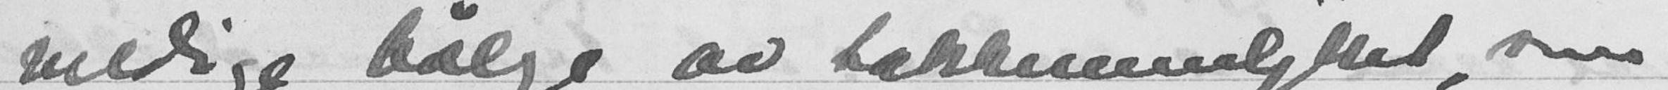veldige bølge av takknemlighet, som
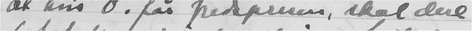at hvis O. fpr fredsprisen, skal dere
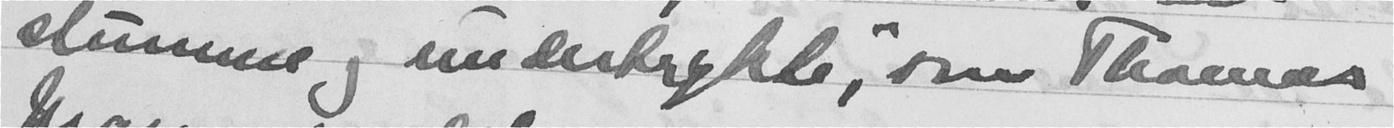stumme og undertrykte, som Thomas
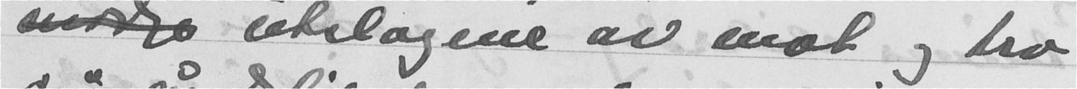utslagene av mot og tro
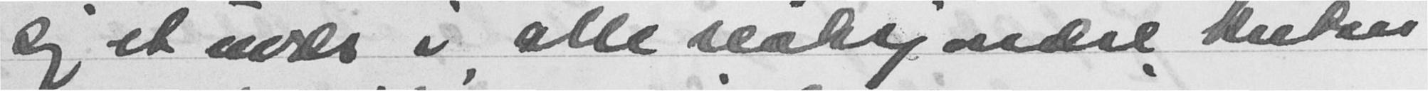sig et uvær i alle reaksjonære kretser
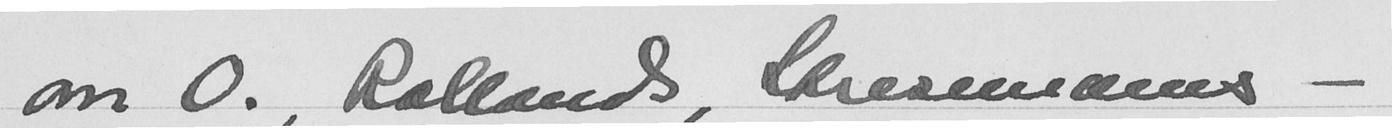om O. Rollands, Skresmanis -
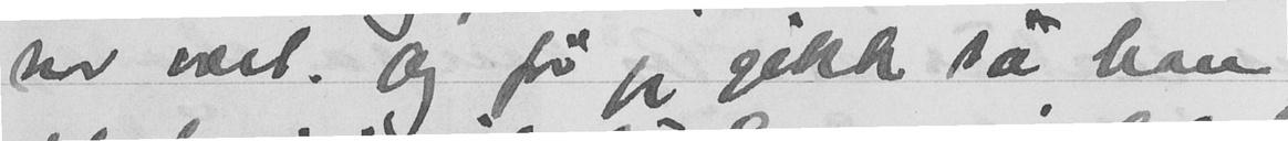har vært, og før jeg gikk sa han
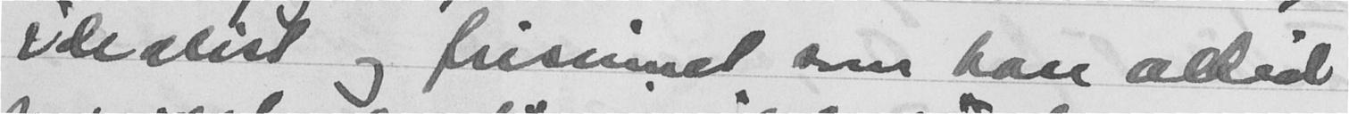idealist og frisinnet som han allid
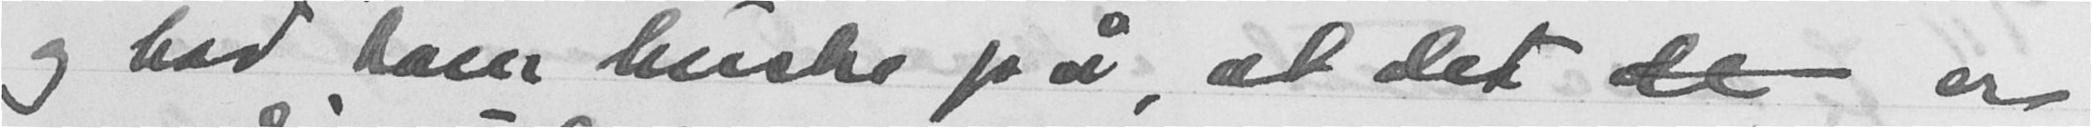og bad ham huske på, at det  er
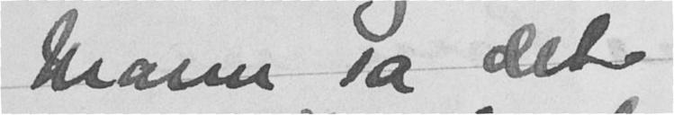Mann sa det.
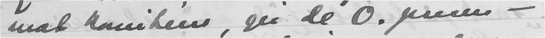mot komitéen, gir de O. prisen -
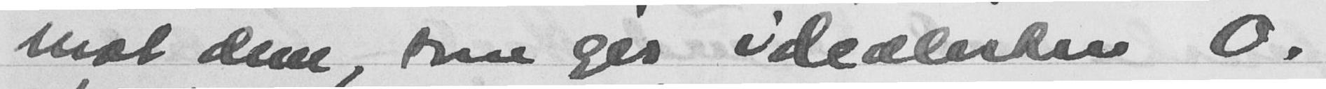mæt dem, som gir idealister O.
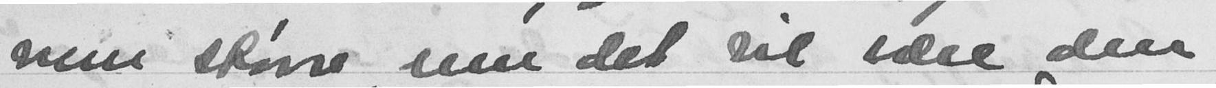men større enn det vil være den
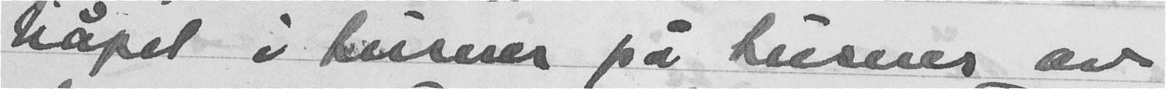håpet i tusener på tusener av
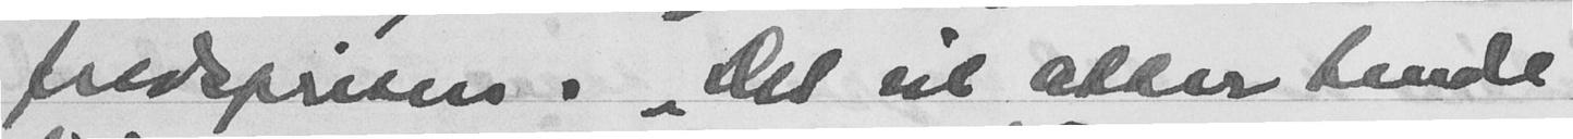fredsprisen. "Det vil atter tende
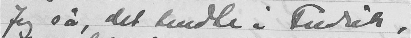Jeg så, det tendte i Fredrik,
